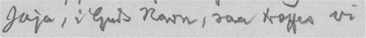Jaja, i Guds Navn, saa træffes vi
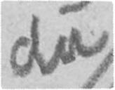du
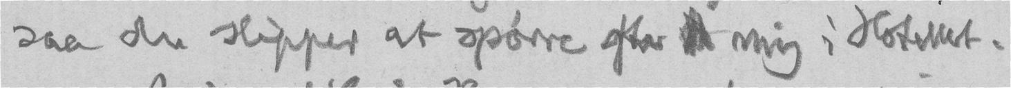saa du slipper at spørre efter  mig i Hotellet.
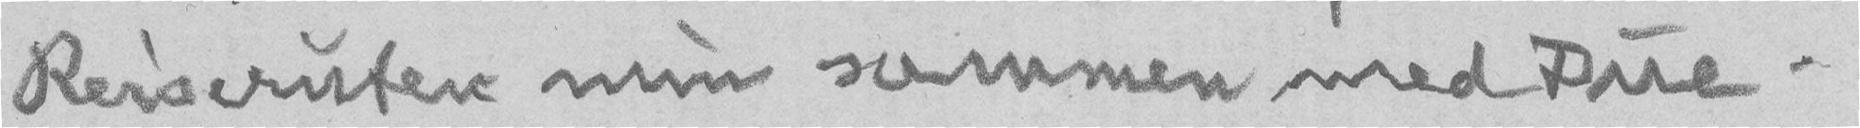Reiseruten min sammen med Due.
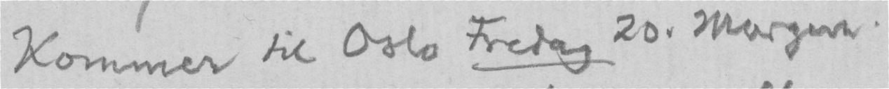Kommer til Oslo Fredag 20. Morgen.
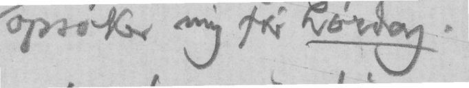opsøker mig før Lørdag.
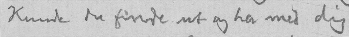Kunde du finde ut og ha med dig
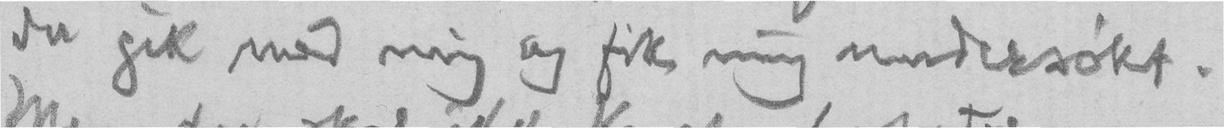du gik med mig og fik mig undersøkt.
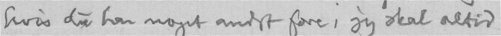hvis du har noget andet fore; jeg skal altid
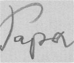Papa
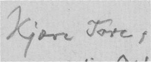Kjære Tore,
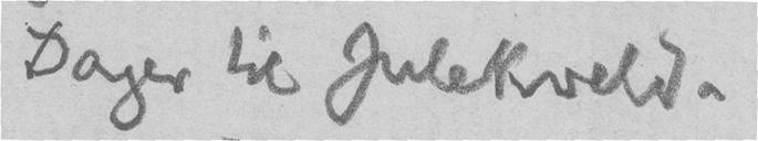Dager til Julekveld.
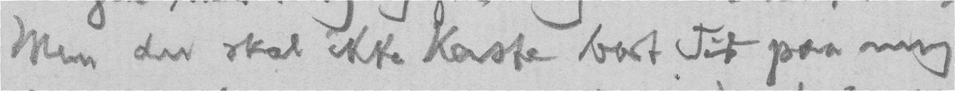Men du skal ikke kaste bort Tid paa mig
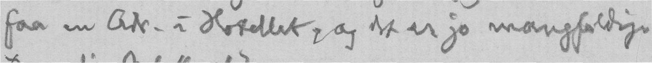faa en Adr. i Hotellet, og det er jo mangfoldige
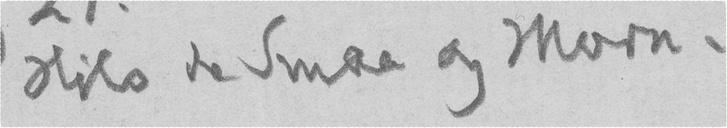Hils de Smaa og Morn.
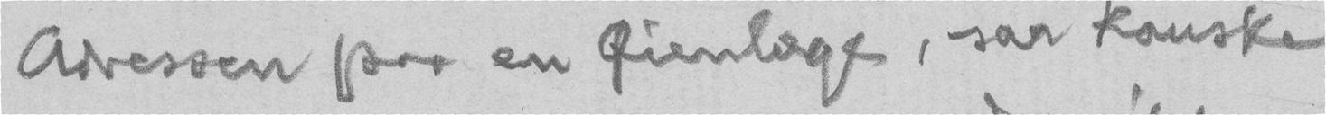Adressen paa en Øienlæge, saa kanske
